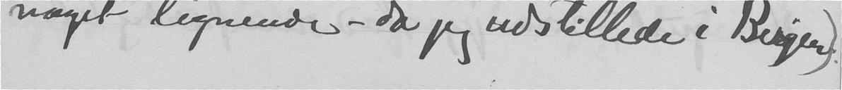noget lignende - da jeg udstillede i Bergen).
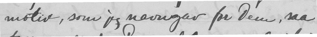motiv, som jeg navngav for Dem, saa
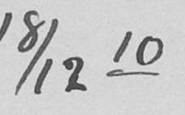18/12-10
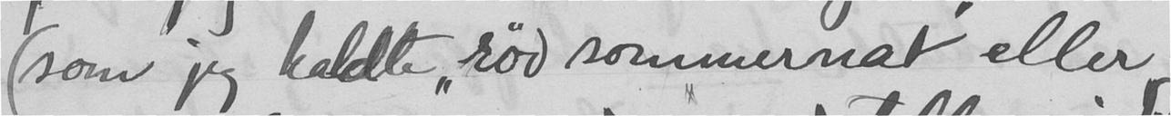(som jeg kaldte "rød sommernat" eller
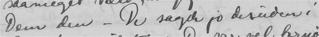Dem den - De sagde jo desuden i
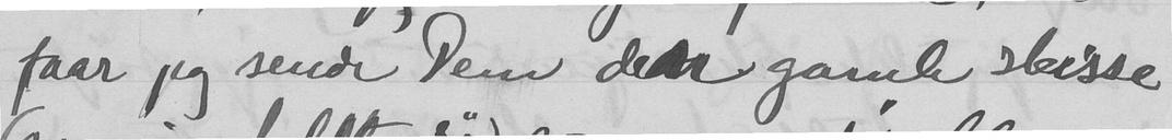faar jeg sende Dem den gamle skisse
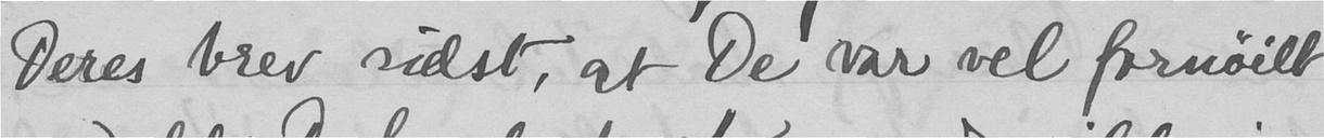Deres brev sidst, at De var vel fornøiet
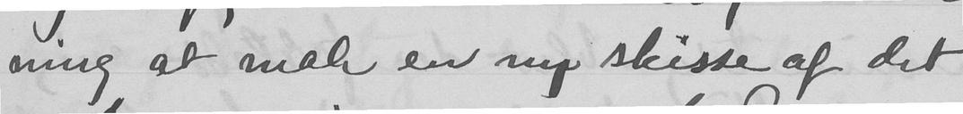ning at male en ny skisse af det
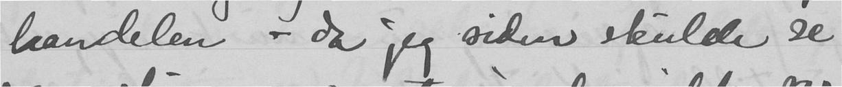handelen - da jeg siden skulde se
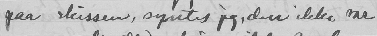paa skissen, syntes jeg, den ikke var
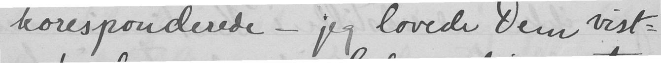koresponderede - jeg lovede Dem vist-
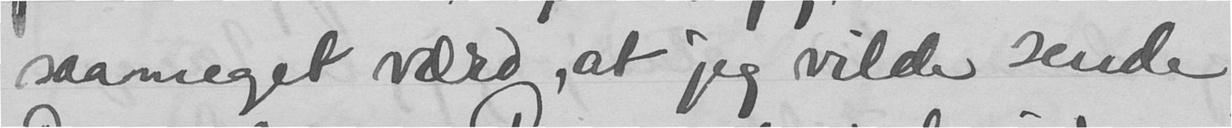saameget værd, at jeg vilde sende
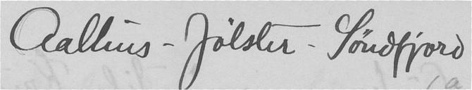Aalhus - Jølster. Søndfjord
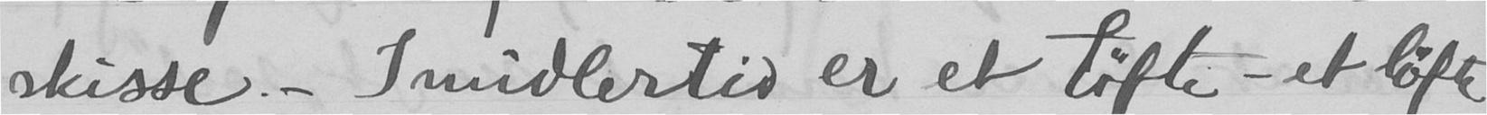skisse. - Imidlertid er et løfte - et løfte
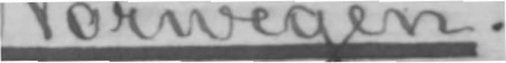Norwegen.
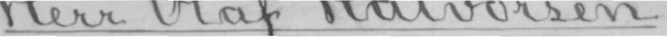Herr Olaf Halvorsen.
